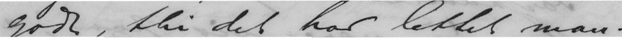godt, thi det har lettet man-
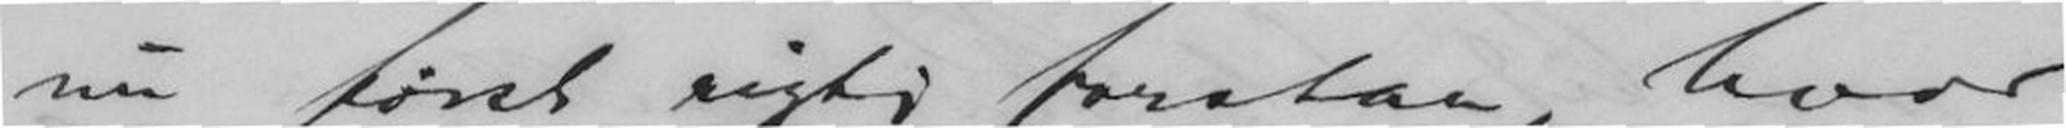nu først rigtig forstaa, hvor
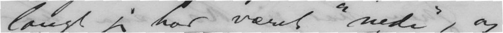langt jeg har været "nede", og
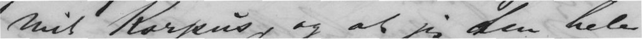mit Korpus, og at jeg den hele
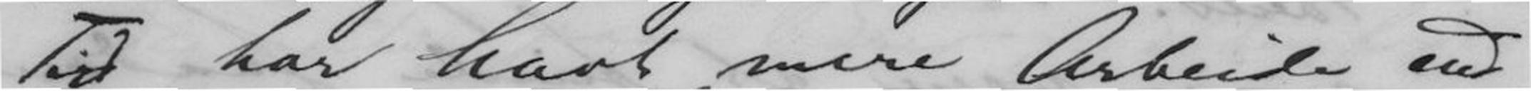Tid har havt mere Arbeide end
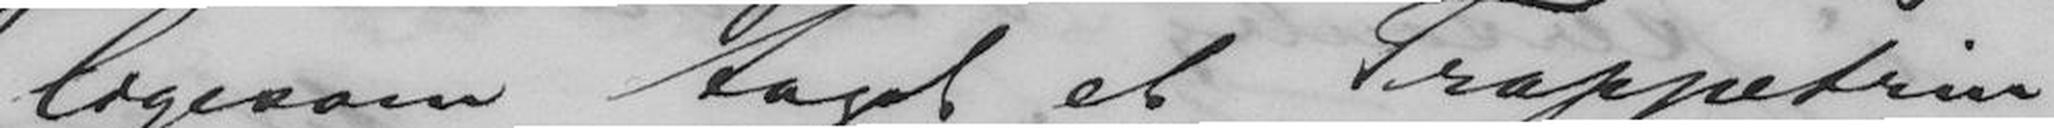ligesom taget et Trappetrin
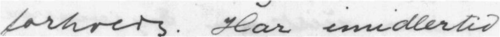forholde. Har imidlertid
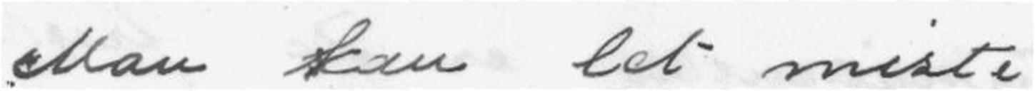Man kan let miste
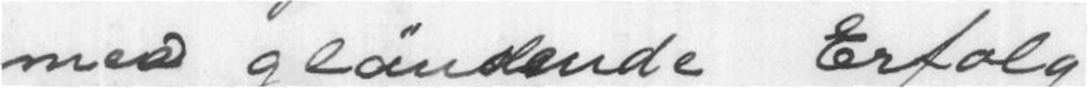med glänzende Erfolg
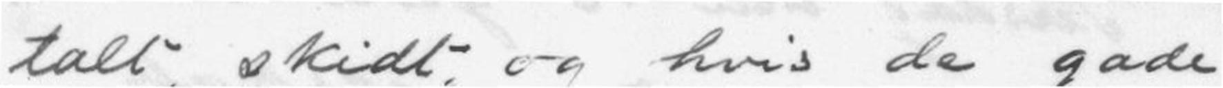talt skidt, og hvis de gode
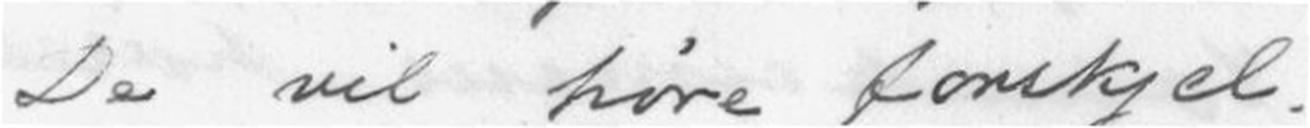De vil høre forskjel.
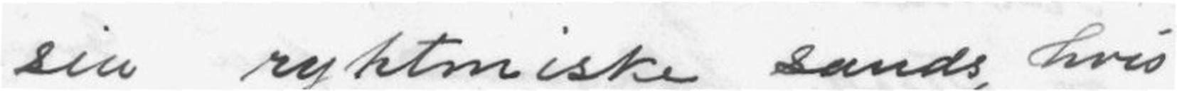sin rytmiske sands, hvis
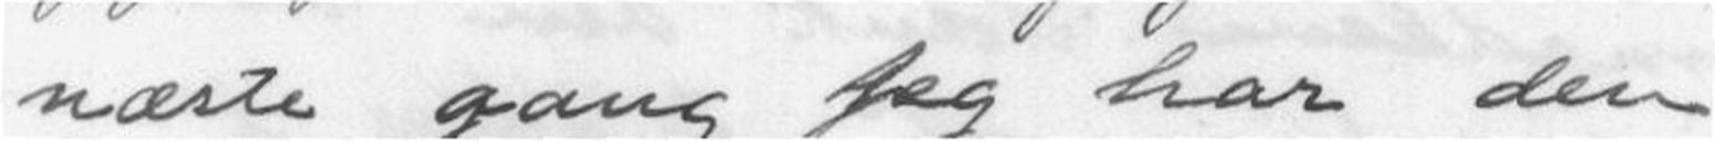næste gang jeg har den
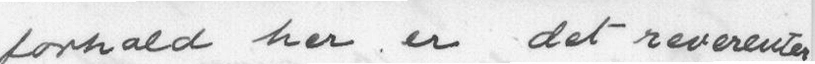forhold her er det reverenter
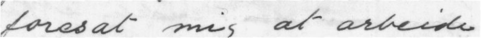foresat mig at arbeide
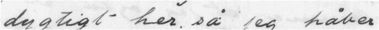dygtigt her, så jeg håber
